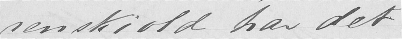renskiold har det
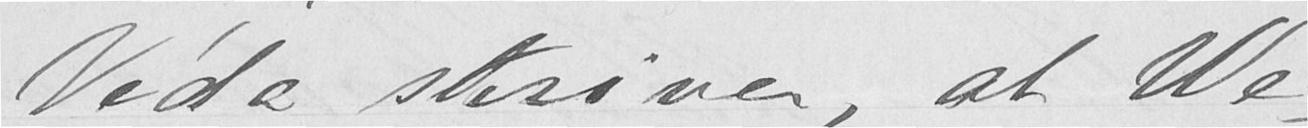Veda skriver, at We-
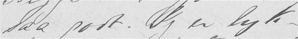saa godt. Jeg er lyk-
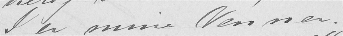I er mine Venner.
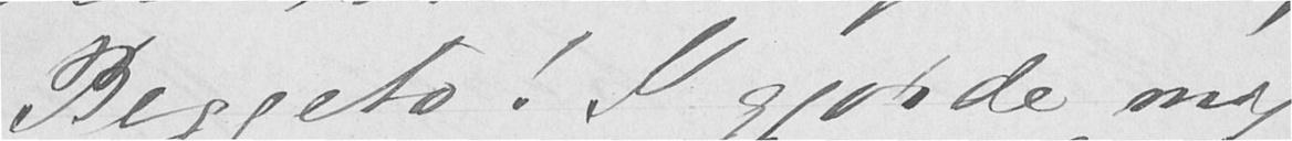Beggeto! I gjorde mig
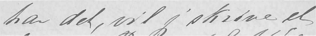har det, vil jeg skrive et
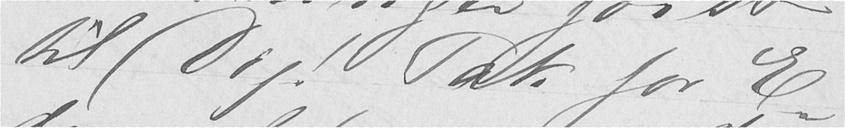til Dig! Tak for E-
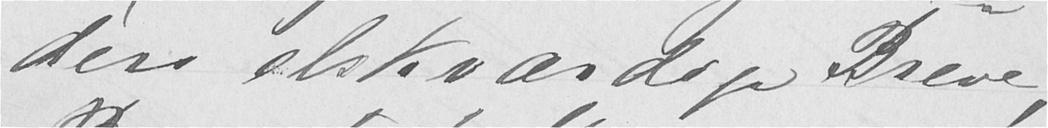ders elskværdige Breve,
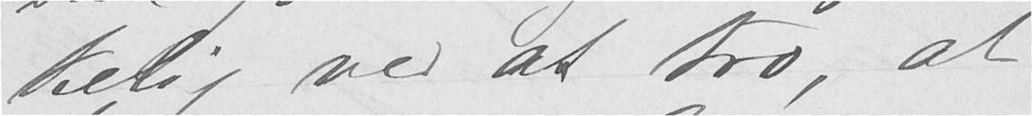kelig ved at tro, at
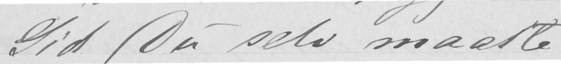Gid Du selv maatte
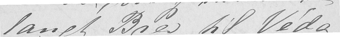langt Brev til Veda.
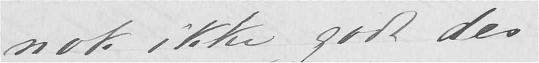nok ikke godt des-
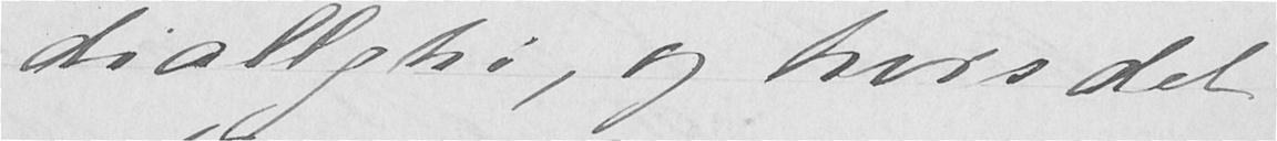diallghi, og hvis det
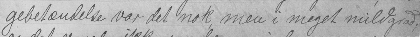gebetændelse var det nok, men i meget mild grad;
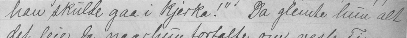han skulde gaa i kjerka!" Da glemte hun alt
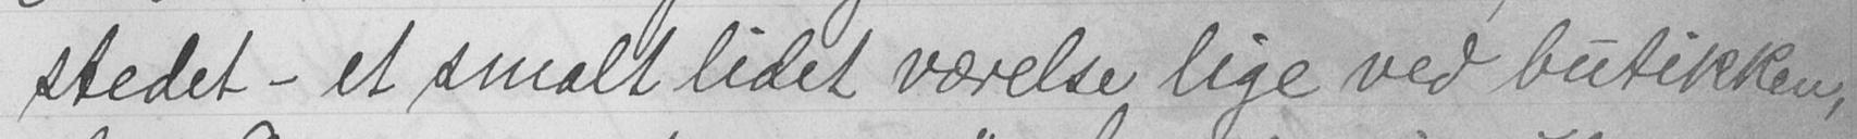stedet - et smalt lidet værelse lige ved butikken,
